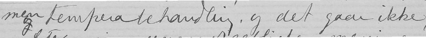men temperabehandling, og det gaar ikke,
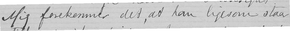Mig forekommer det, at han ligesom staar
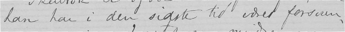han har i den sidste tid været forsvun-
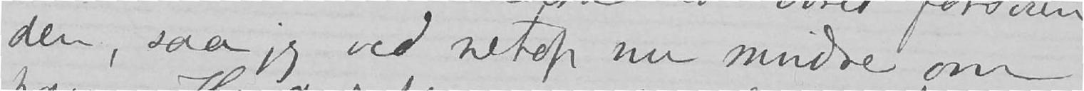den, saa jeg ved netop nu mindre om
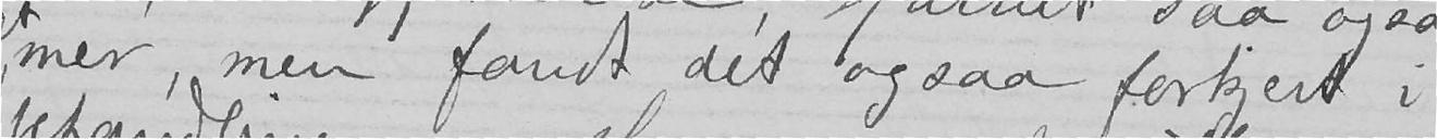mer, men fandt det ogsaa forkjert i
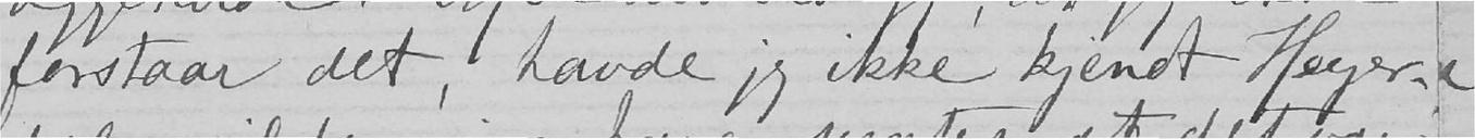forstaar det, havde jeg ikke kjendt Heyer-
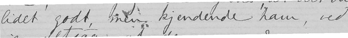lidet godt, men kjendende ham, ved
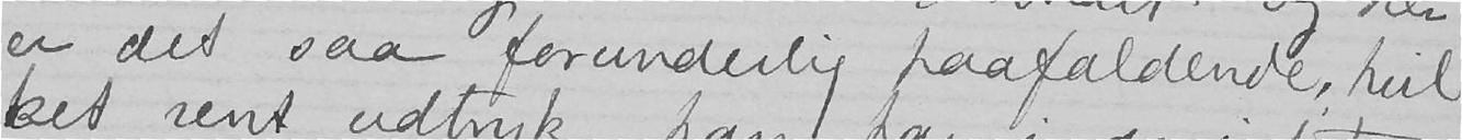er det saa forunderlig paafaldende, hvil-
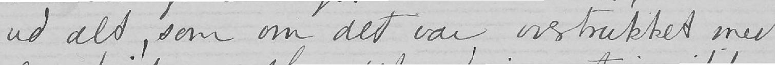ud alt, som om det var overtrukket med
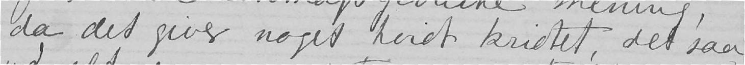da det giver noget hvidt kridtet, det saa
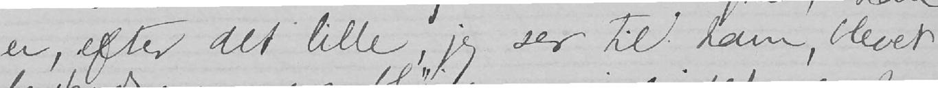er, efter det lille, jeg ser til ham, blevet
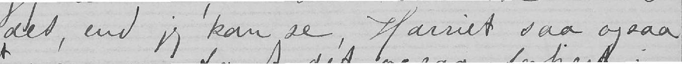det, end jeg kan se, Harriet saa ogsaa
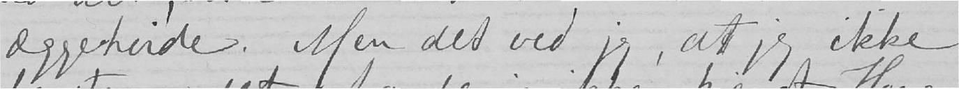æggehvide. Men det ved jeg, at jeg ikke
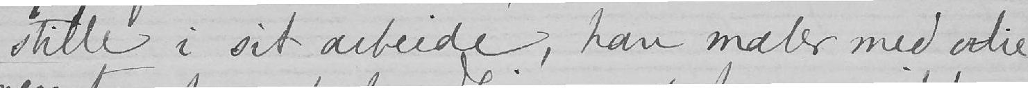stille i sit arbeide, han maler med olie
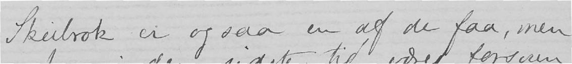Skeibrok er ogsaa en af de faa, men
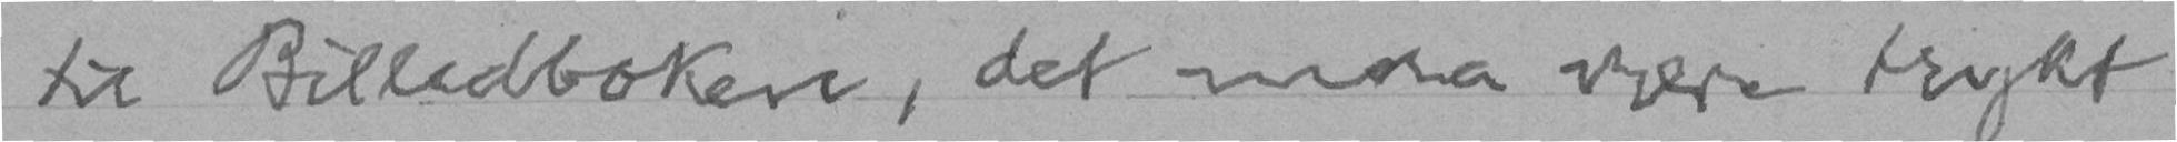til Billedboken, det maa være trykt
